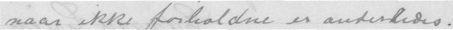naar ikke forholdne er anderledes.
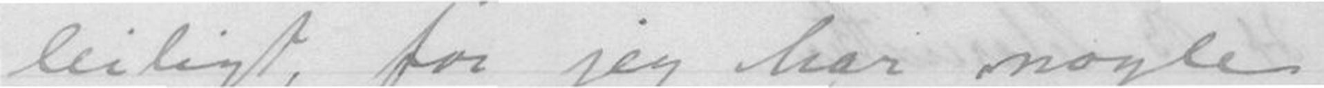leiligt, for jeg har nogle
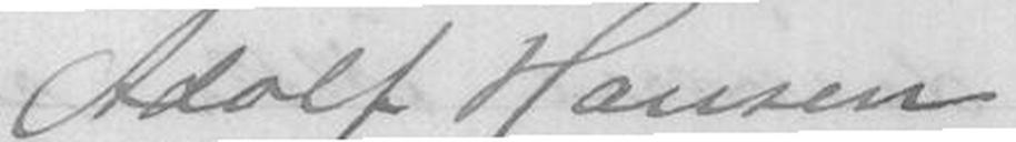Adolf Hansen
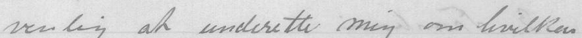venlig at underette mig om hvilken
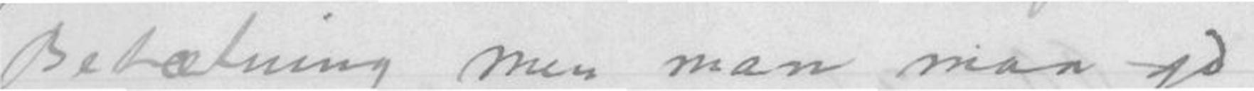Besætning men man maa jo
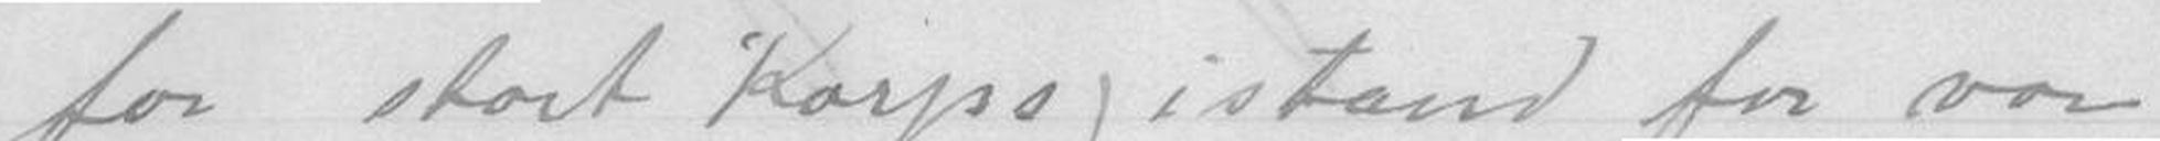for stort Korps) istand for vor
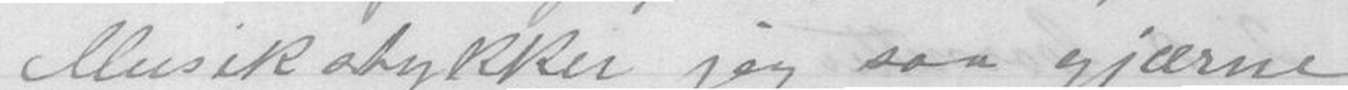Musikkstykker jeg saa gjærne
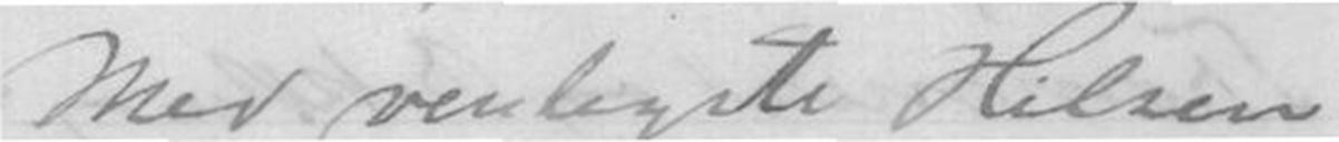Med venlegste Hilsen
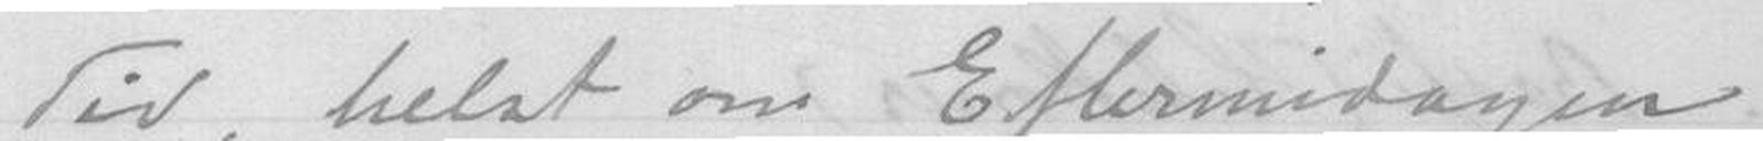tid, helst om Eftermidagen
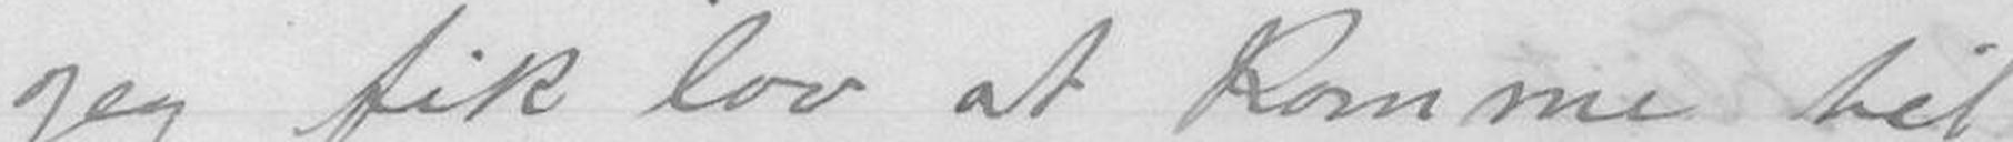jeg fik lov at komme til
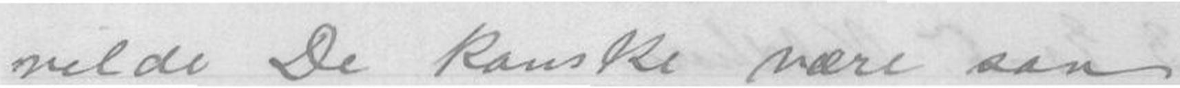vilde De kanske være saa
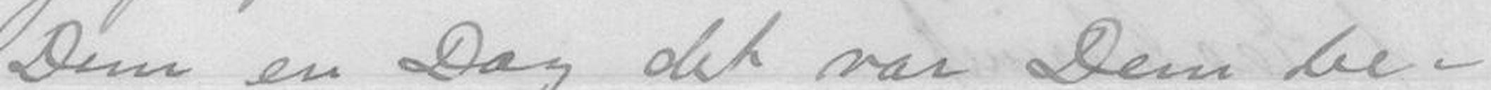Dem en Dag det var Dem be-
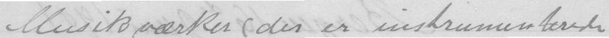Musikværker (der er instrumenterede
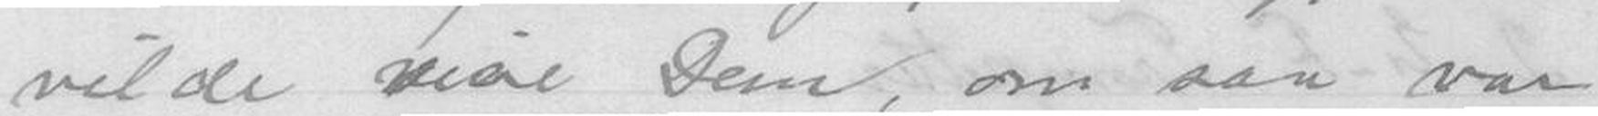vilde vise Dem, om saa var
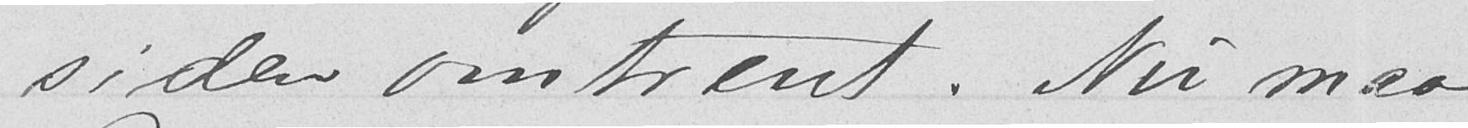siden omtrent. Nu maa
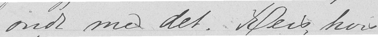ondt med det. Reis hvis
